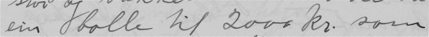ein bolle til 2000 kr. som
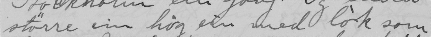större ein med hög ein med lok som
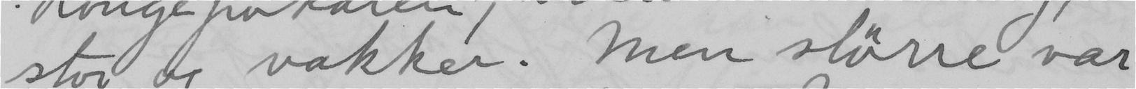stor og vakker. Men större var
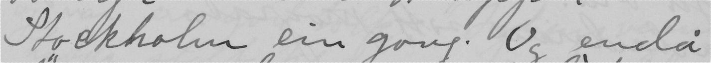Stockholm ein gang. Og endå
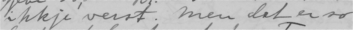ikkje verst. Men det er so
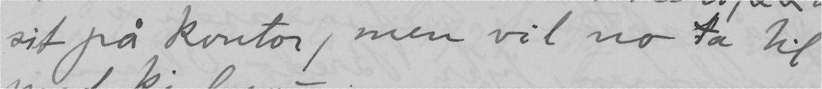sit på kontor, men vil no ta til
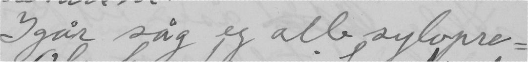Igår såg eg alle sylvpremiane
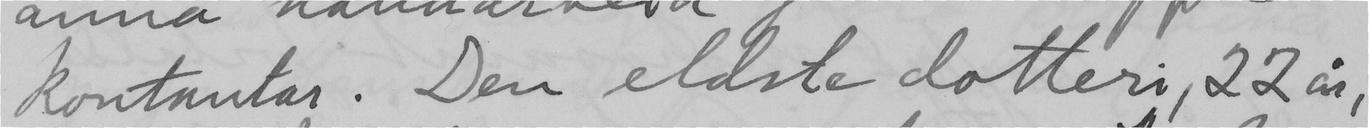kontantar. Den eldste dotteri, 22 år,
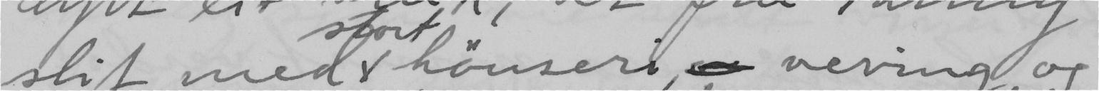slit med stort hönseri, veving og
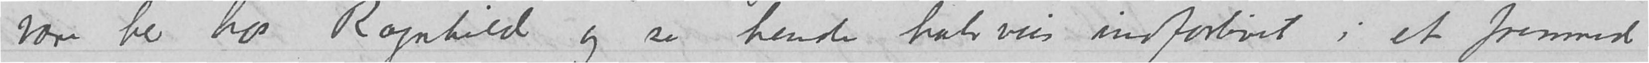være her hos Ragnhild og se hende halvveis indforlivet i et fremmed
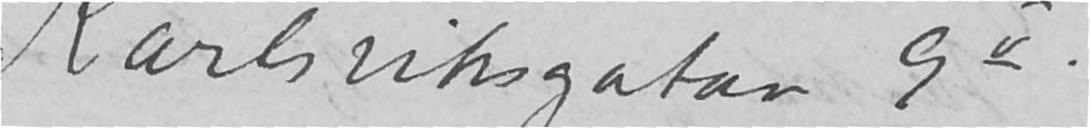Karlsvikgatan 9 v.
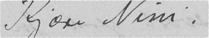Kjære Nini,
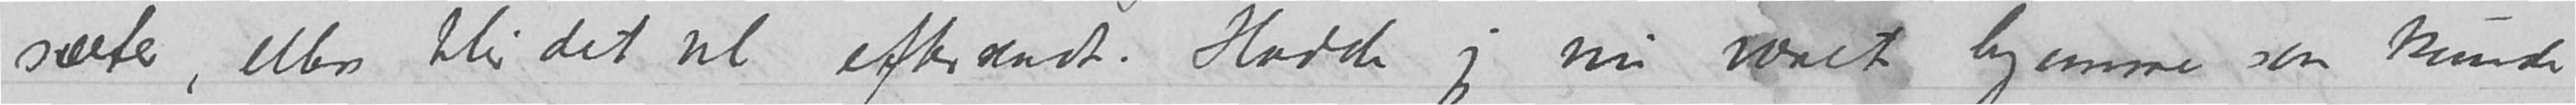sæter, ellers blir det vel eftersendt.  Hadde jeg nu været hjemme saa kunde
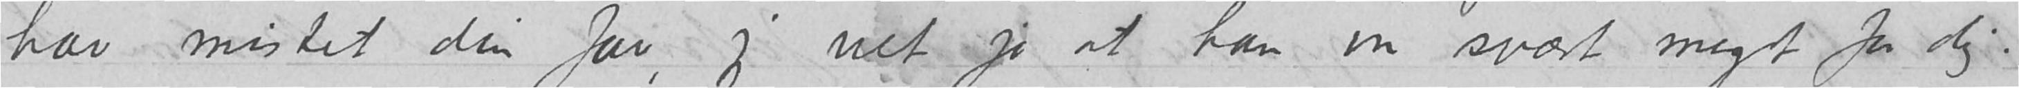har mistet din far, jeg vet jo at han var svært meget for dig.
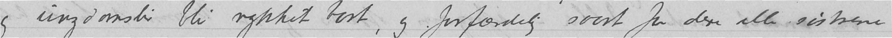og ungdomsliv blir rykket bort, og forfærdelig saart for dere alle søstrene
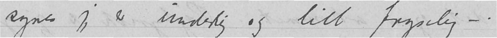synes jeg er underlig og litt fryselig –.
